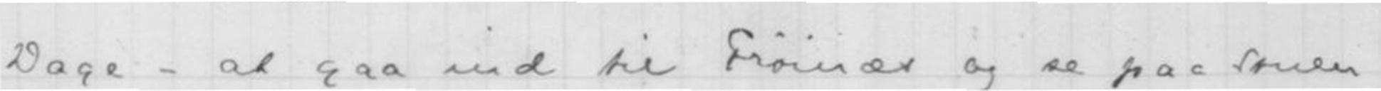Dage - at gaa ind til Frøinæs og se paa Stuen
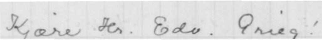Kjære Hr. Edv. Grieg!
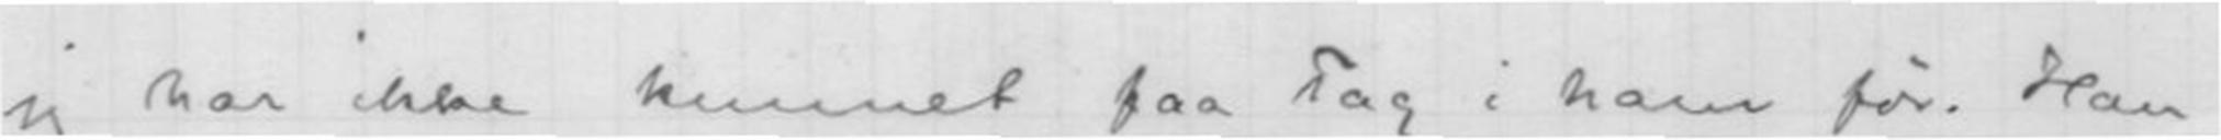jeg har ikke kunnet faa Tag i ham før. Han
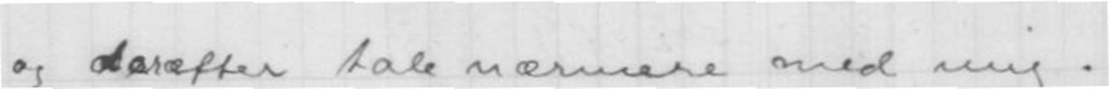og deræfter tale nærmere med mig.
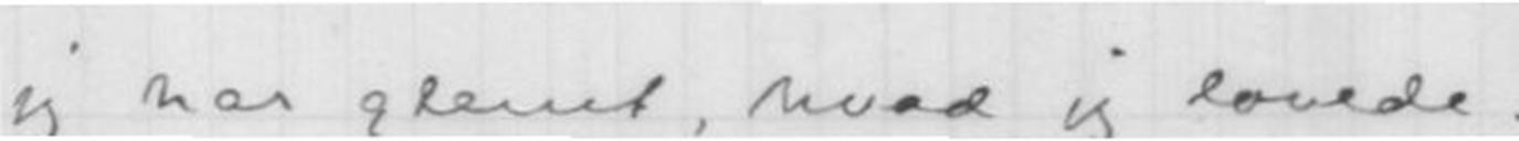jeg har glemt, hvad jeg lovede.
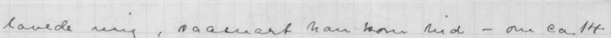lovede mig, saasnart han kom hid - om ca. 14
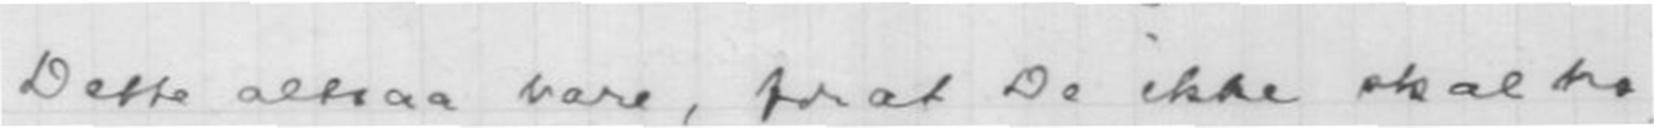Dette altsaa bare, for at De ikke skal tro,
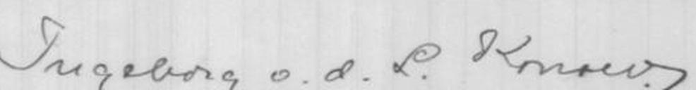Ingeborg o.d. L. Konow.
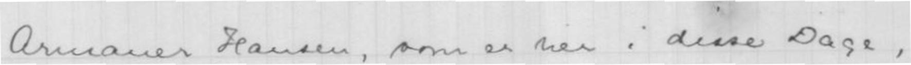Armauer Hansen, som er her i disse Dage,
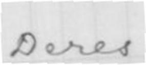Deres
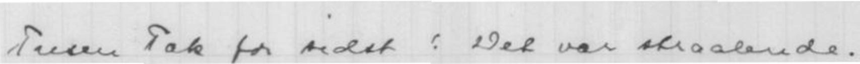Tusen Tak for sidst: Det var straalende.
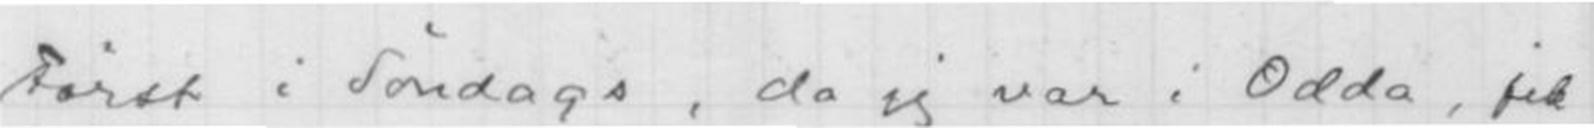Først i Søndags, da jeg var i Odda, fik
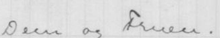Dem og Fruen.
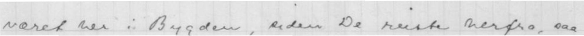været her i Bygden, siden De reiste herfra, saa
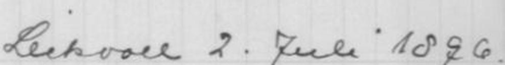Leikvoll 2. Juli 1896.
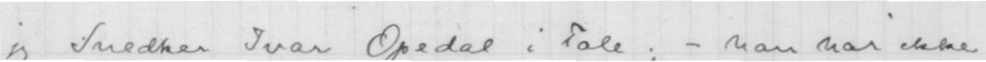jeg Snedker Ivar Opedal i Tale; - han har ikke
